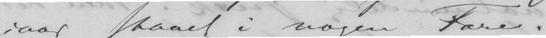iaar staaet i nogen Fare.
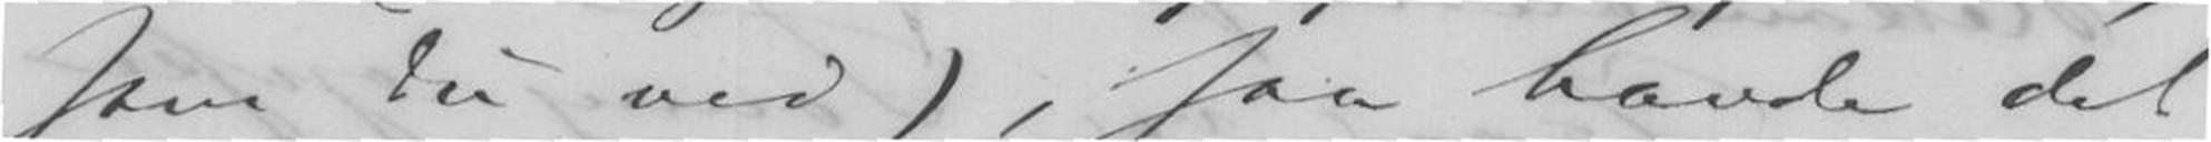som du ved), saa havde det
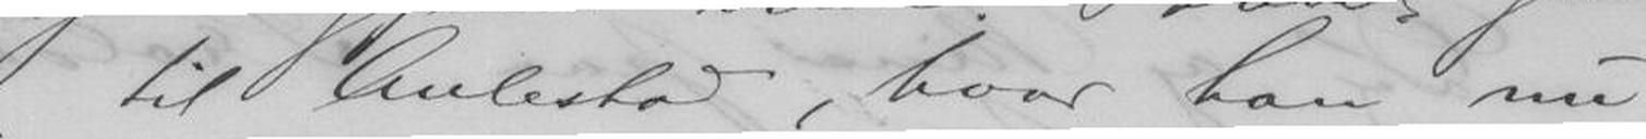jeg til Aulestad, hvor han nu
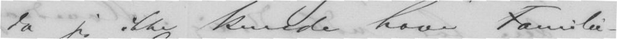da jeg ikke kunde have Familie-
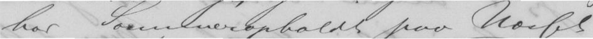har Sommeropholdet paa Næsset
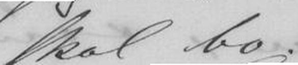skal bo.
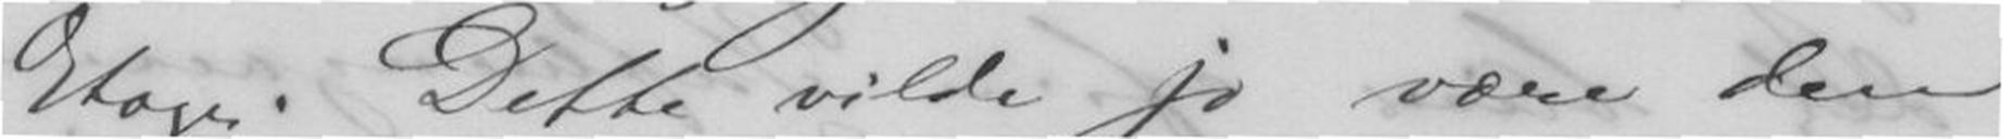Etage. Dette vilde jo være den
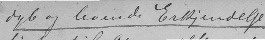dyb og levende Erkjendelse,
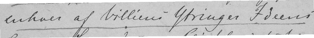enhver af Villiens Ytringer Ideens
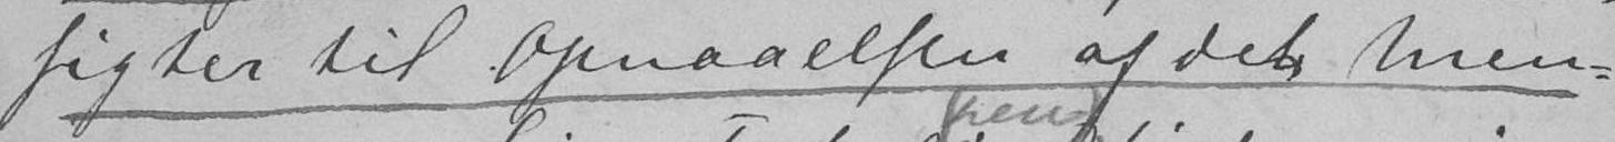sigter til Opnaaelsen af det men-
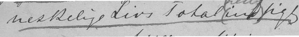neskelige Livs Totalindsigt
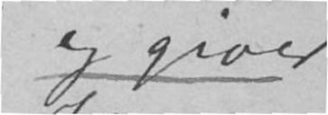og giver
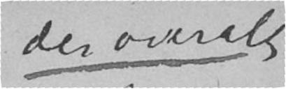der overalt
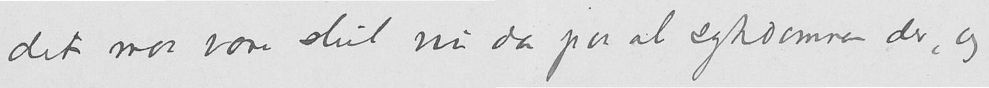det maa være slut nu da paa al sykdommen der, og
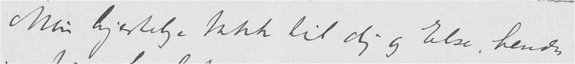Min hjertelige takk til dig og Else, hendes
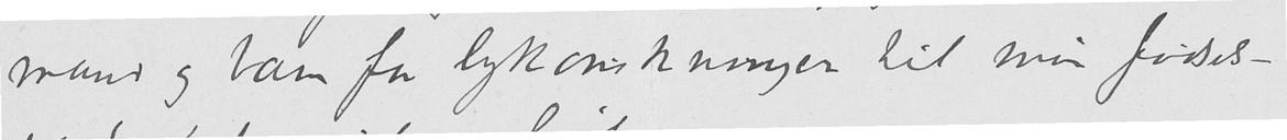mand og barn for lykønskninger til min fødsels-
